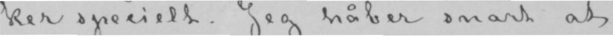ker specielt. Jeg håber snart at
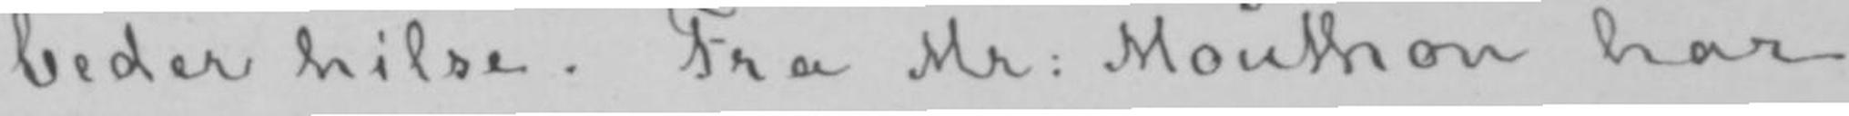beder hilse. Fra Mr: Mouthon har
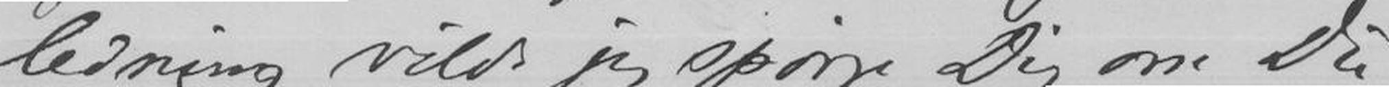ledning vilde jeg spørge Dig om Du
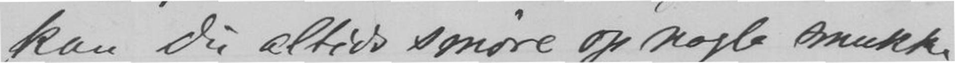kan Du altids smøre op nogle smukke
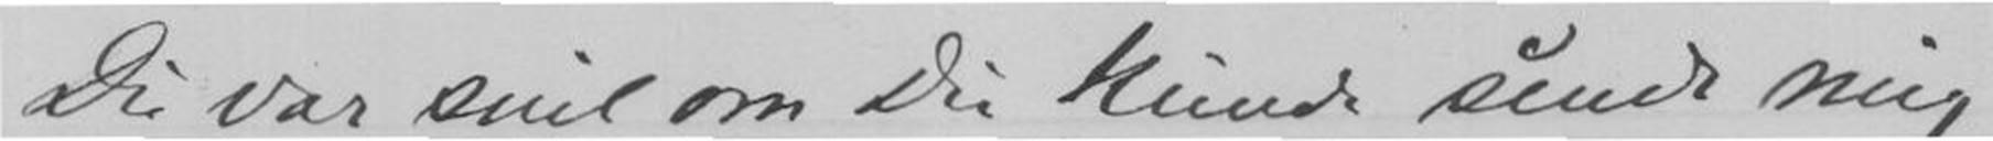Du var snil om Du kunde sende mig
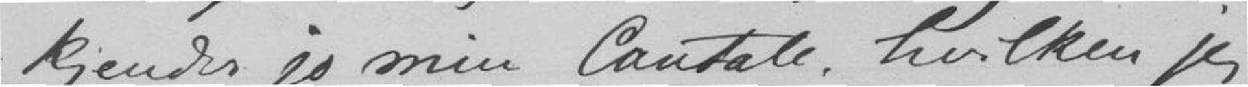kjendes jo min Contak, hvilken jeg
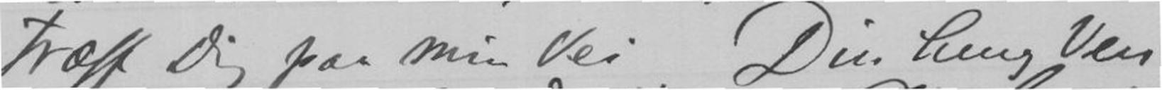træff Dig paa min Vei.Din heng Ven
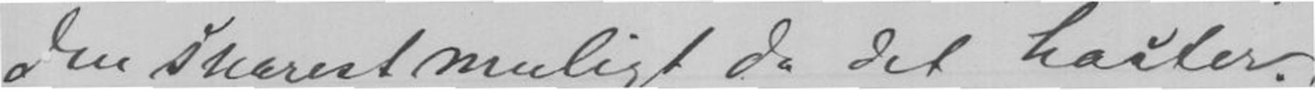den snarest muligt da det haster.
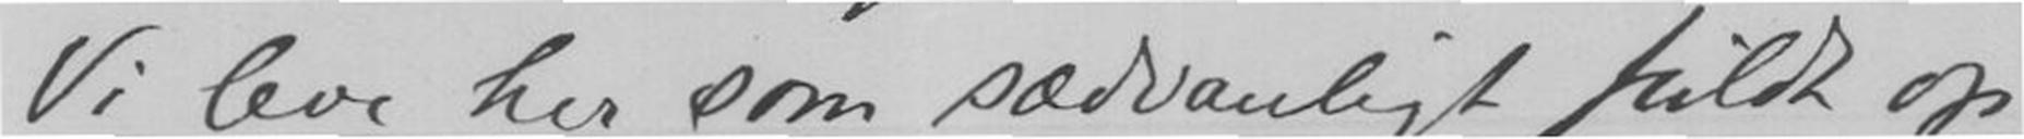Vi leve her som sædvanligt fuldt op
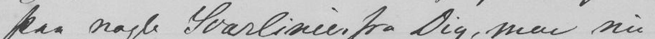paa nogle Svarlinie fra Dig men nu
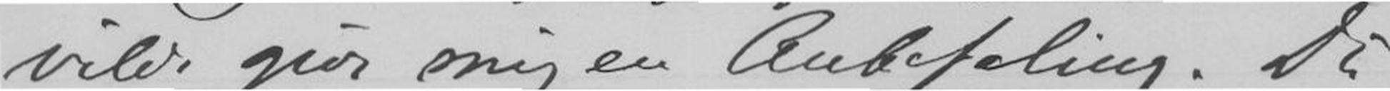vilde give mig en Anbefaling. Du
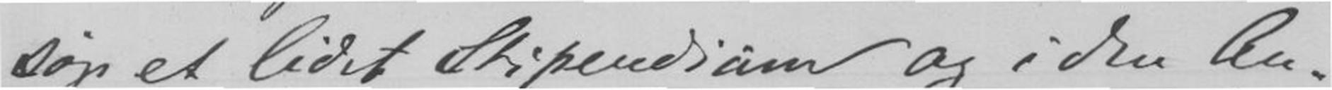søge et lidet Stipendium og i den An-
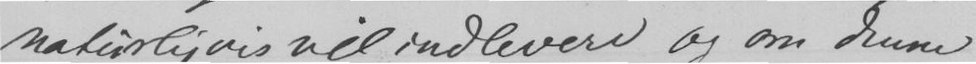naturligvis vil indlevere og om denne
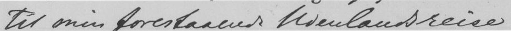til min forestaaende Udenlandsreise
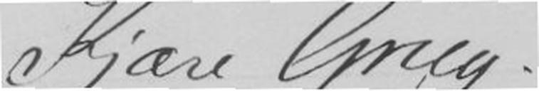Kjære Grieg!
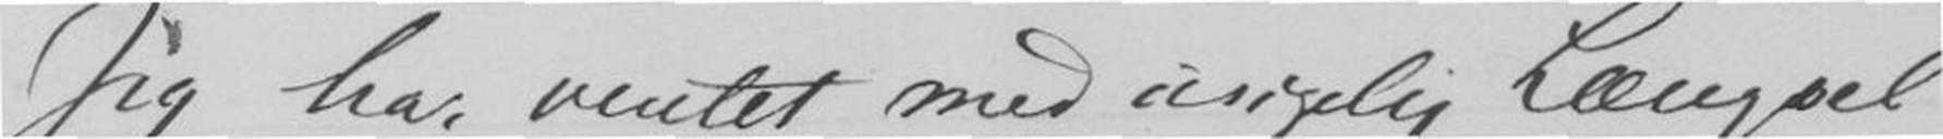Jeg har ventet med usigelig Længsel
